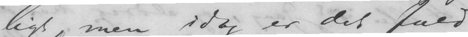ligt, men idag er det fuld
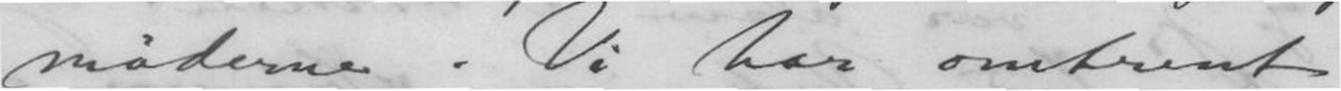møderne. Vi har omtrent
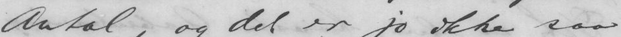Antal, og det er jo ikke saa
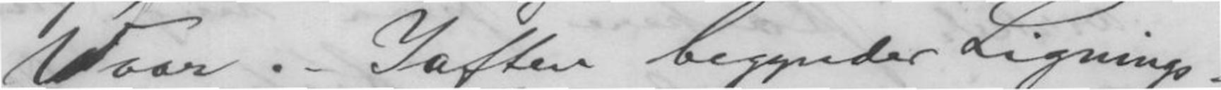Vaar. - Iaften begynder Lignings-
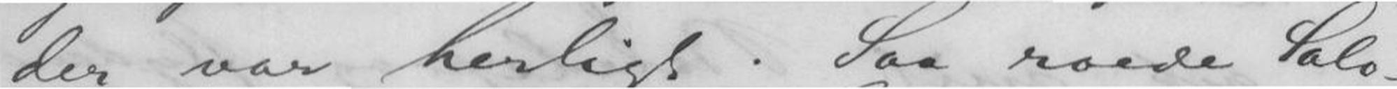der var herligt. Saa roede Salo-
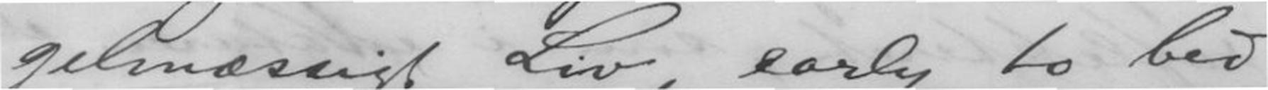gelmæssigt Liv. early to bed
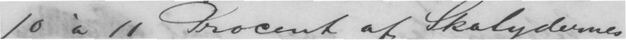10 à 11 Procent af Skatydernes
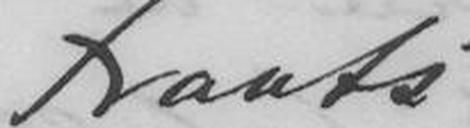Frants.
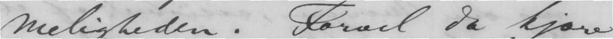meligheden. Farvel da kjære
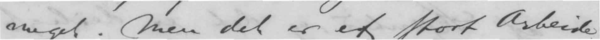meget. Men det er et stort Arbeide,
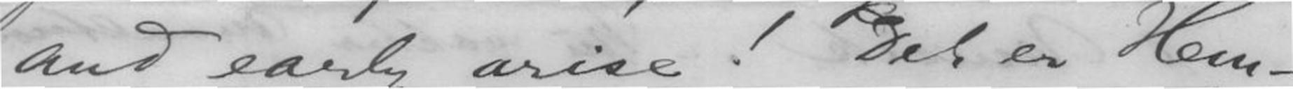and early arise! Det er Hem-
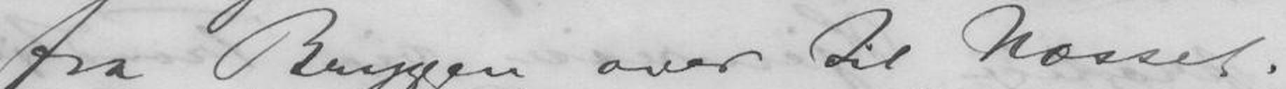fra Bryggen over til Næsset.
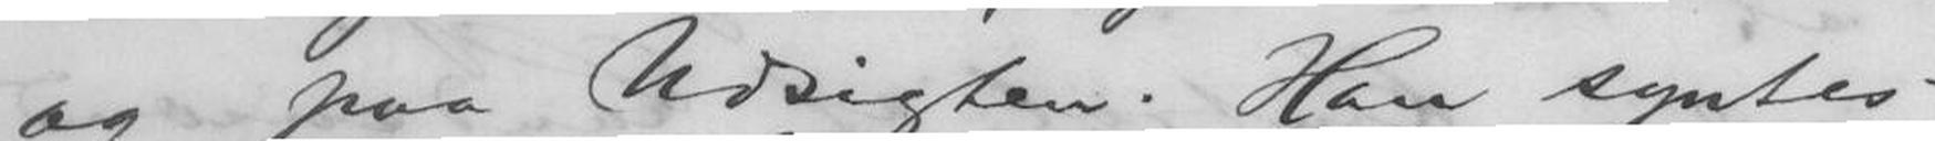og paa Udsigten. Han syntes
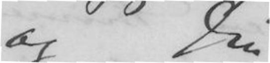og Din
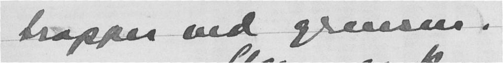trapper ved grensen.
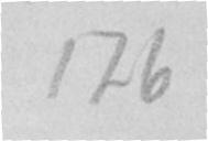176
